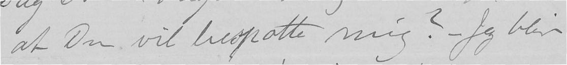at Du vil bespotte mig? - Jeg blir
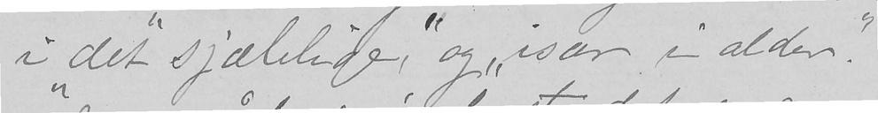i "det sjælelige", og "især i alder".
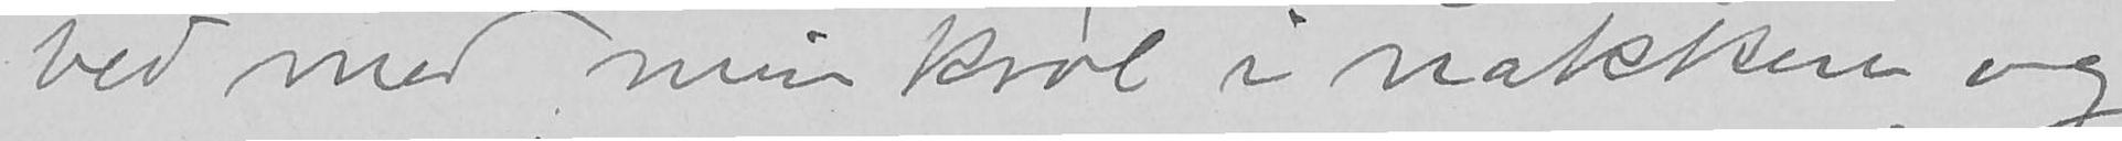ved med min krøl i nakken og
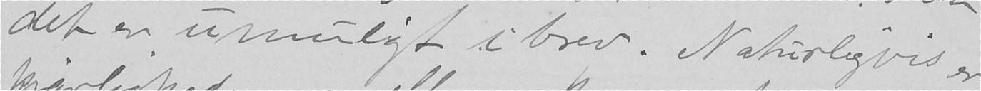det er umuligt i brev. Naturligvis er
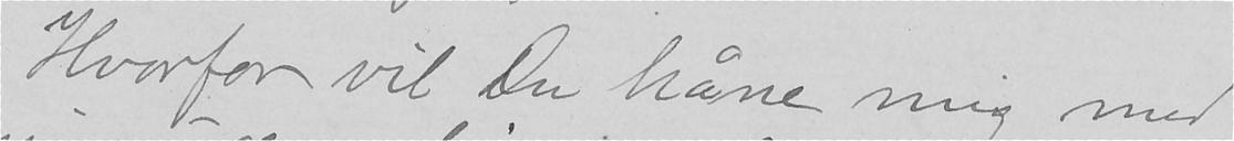Hvorfor vil du håne mig med
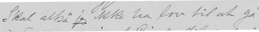Skal altså jeg ikke ha lov til at gå
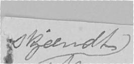skjendt
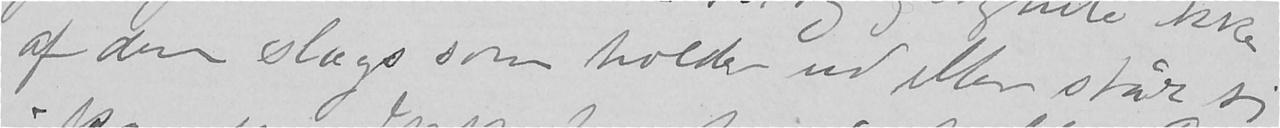af den slags som holder ud eller står sig
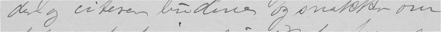der og citerer budene og snakker om
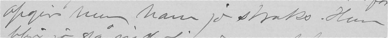opgir hun han jo straks. Hun
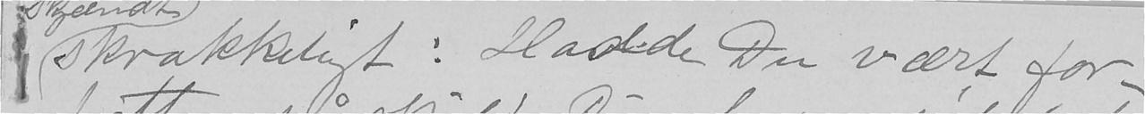skrækkeligt: Hadde Du vært for-
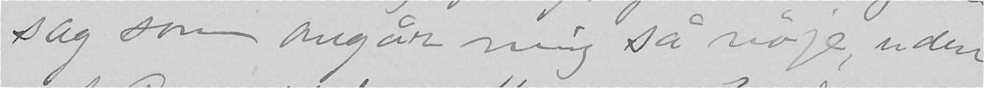sag som angår mig så nøje uden
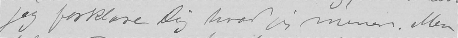jeg forklare Dig hvad jeg mener. Men
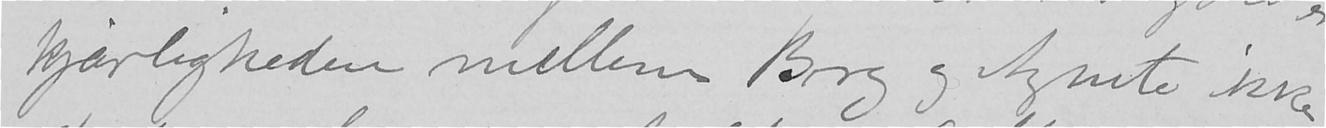kjærligheden mellem Berg og Agnete ikke
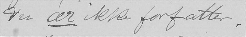Du ær ikke forfatter.
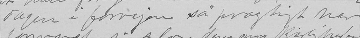dagen i forvejen så prægtigt har
